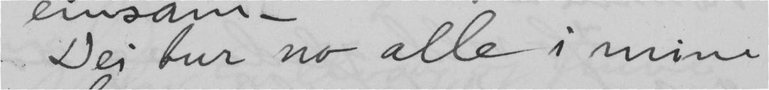Der bur no alle i mine
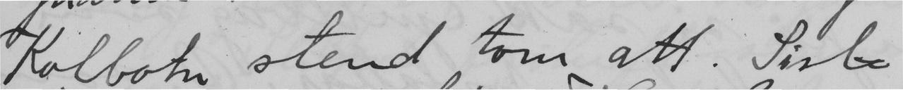Kolbotn stend tom att. Siste
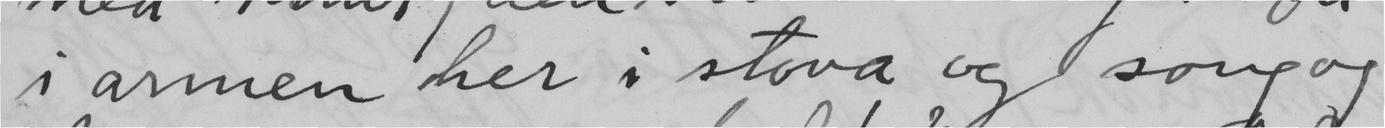i armen her i stova og song og
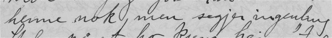henne nok, men segjer ingenting
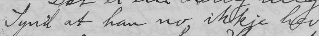Synd at han no ikkje hev
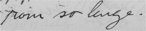rom so lenge.
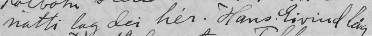natti lag dei her. Hans Eivind låg
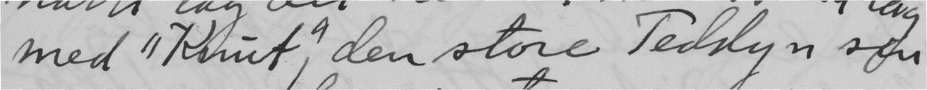med "Knut", den store Teddy'n sin
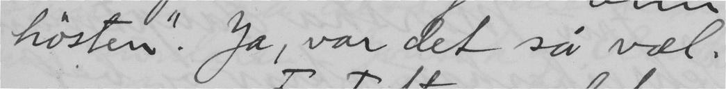hösten". Ja, var det så væl.
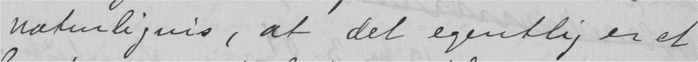naturligvis, at det egentlig er et
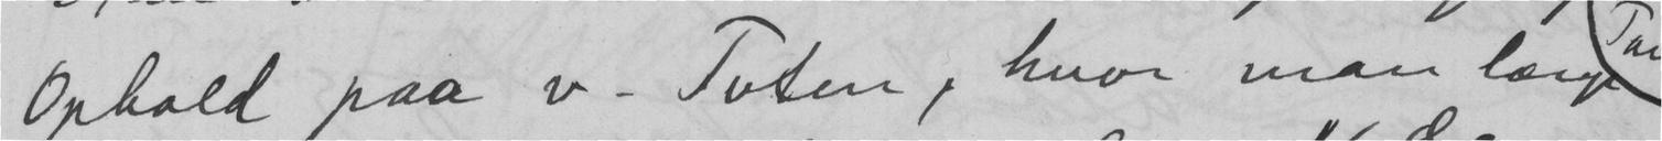Ophold paa v. Toten, hvor man længe
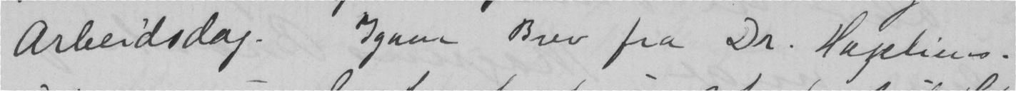arbeidsdag. Igaar Brev fra Dr. Hagelius.
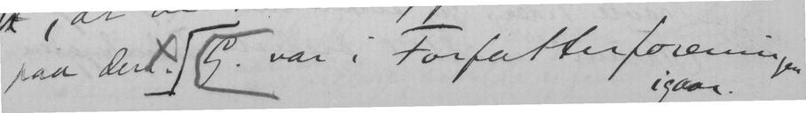paa den. G. var i Forfatterforenm.
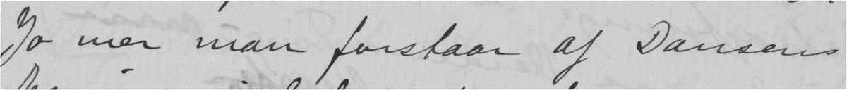Jo mer man forstaar af Dansens
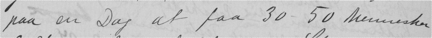paa en Dag at faa 30 - 50 Mennesker
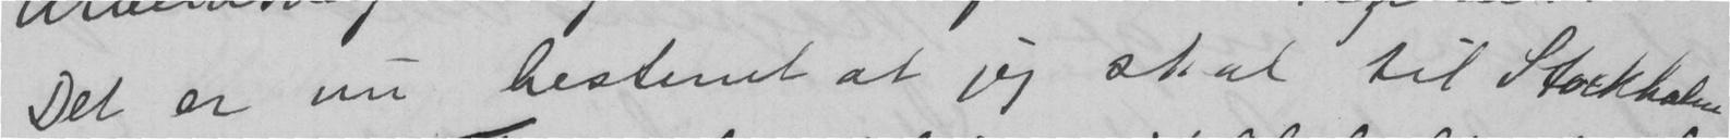Det er nu bestemt at jeg skal til Stockholm
